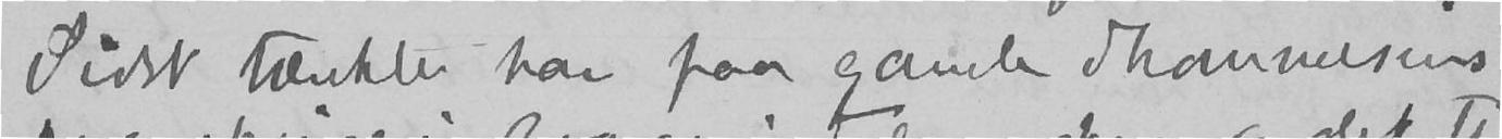Sidst tænkte han paa gamle Thommessens
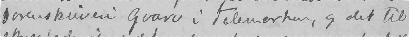Sorenskriveri Gvarv i Telemarken, og det til-
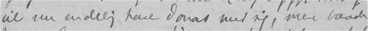vil nu endelig have Jonas med sig, men baade
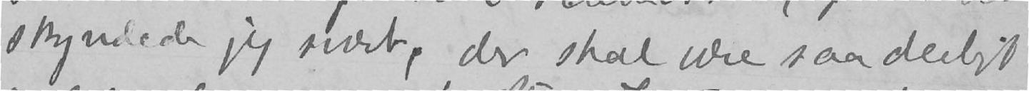skyndede jeg svært, der skal være saa deiligt
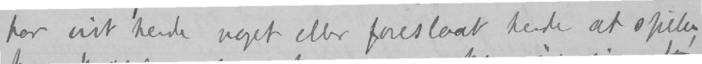har vist hende noget eller foreslaat hende at spille,
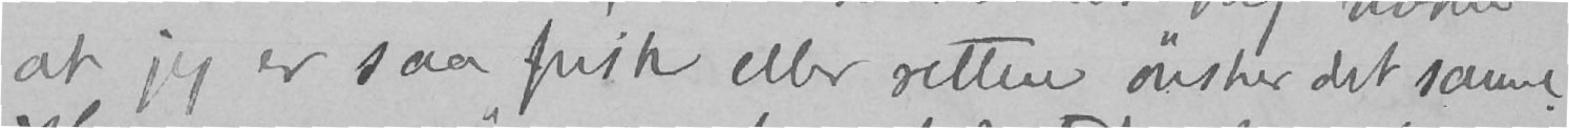at jeg er saa frisk eller rettere ønsker det samme.
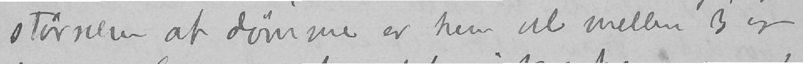størrelsen at dømme er hun vel mellem 3 og
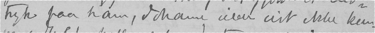tryk paa ham, Johanne vilde vist ikke kun-
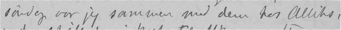søndag var jeg sammen med dem hos Alliks,
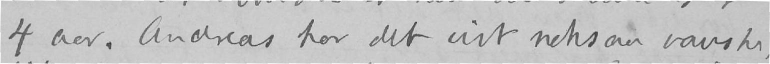4 aar. Andreas har det vist noksaa vanske-
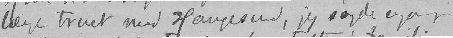længe truet med Haugesund, jeg sagde engang
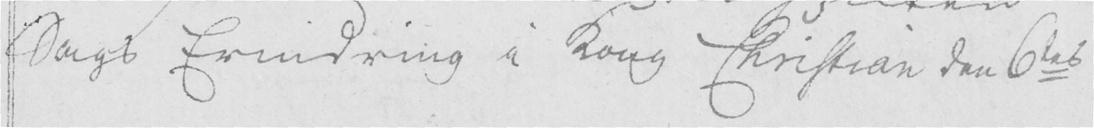Sags Erindring i Kong Christian den 6tes
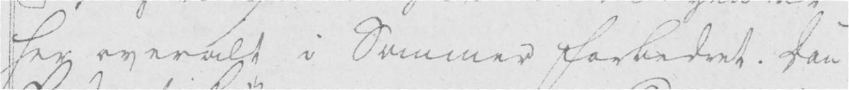her overalt i Sommer forbedret. Den
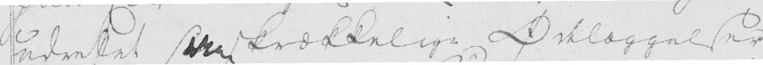udrettet saa skrækkelige Ødelæggelser
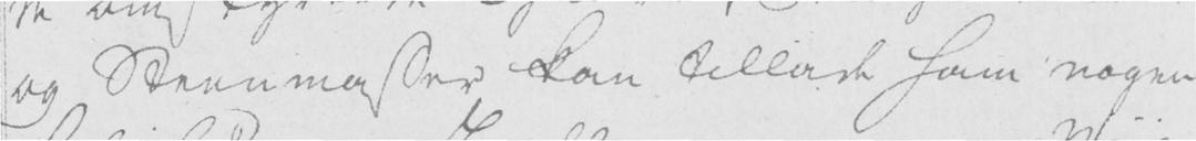og Steenmasser kan tillade ham nogen
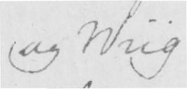og Wiig
